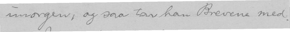imorgen, og saa tar han Brevene med.
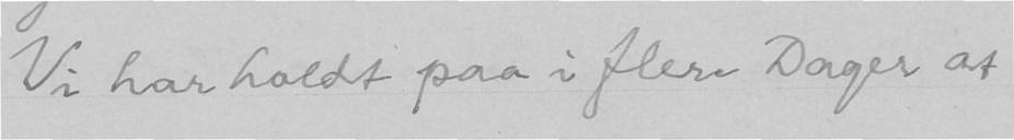Vi har holdt paa i flere Dager at
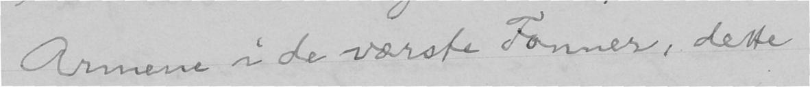Armene i de værste Fonner, dette
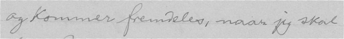og kommer fremdeles, naar jeg skal
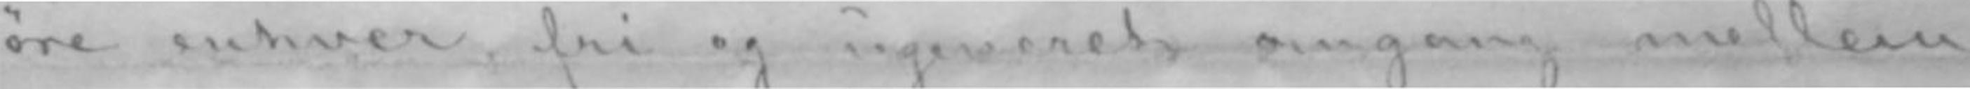gøre enhver fri og ugeneret omgang mellem
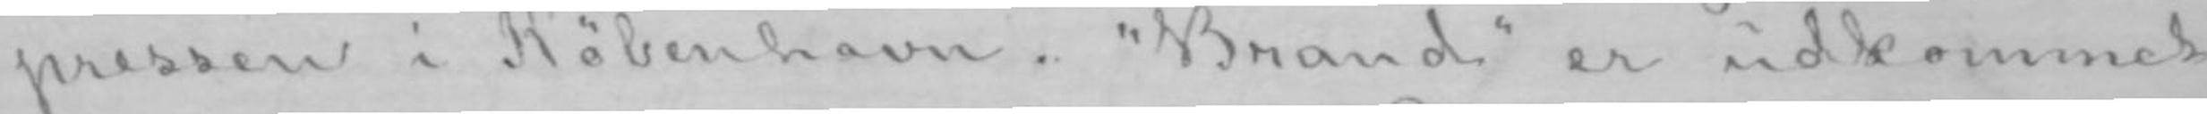pressen i København. «Brand» er udkommet
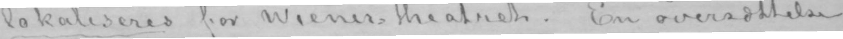lokaliseres for Wiener-theatret. En oversættelse
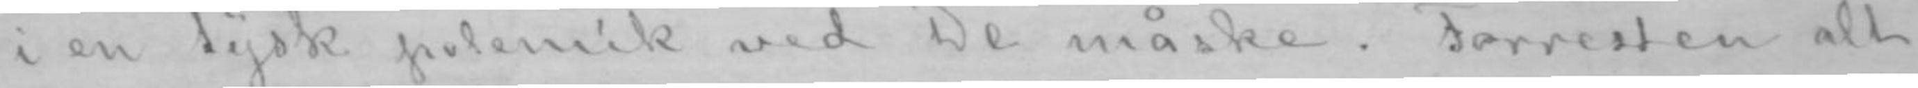i en tysk polemik ved De måske. Forresten alt
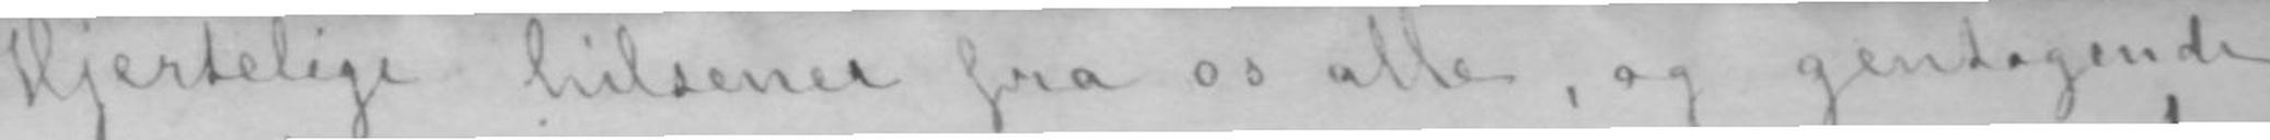Hjertelige hilsener fra os alle, og gentagende
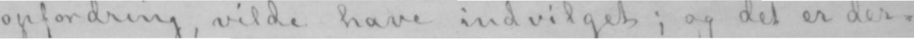på opfordring, vilde have indvilget; og det er der-
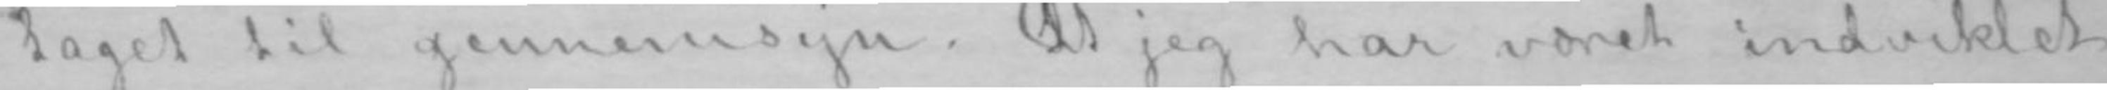taget til gennemsyn. At jeg har været indviklet
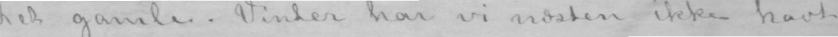ved det gamle. Vinter har vi næsten ikke havt
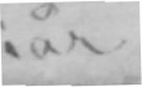iår.
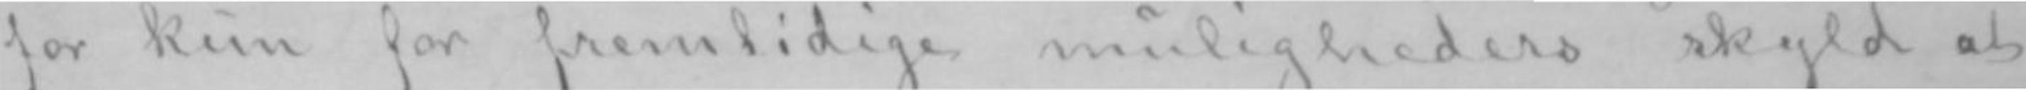for kun for fremtidige muligheders skyld at
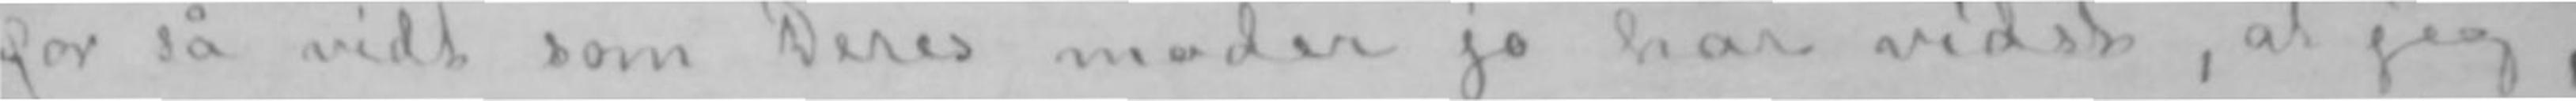for så vidt som Deres moder jo har vidst, at jeg,
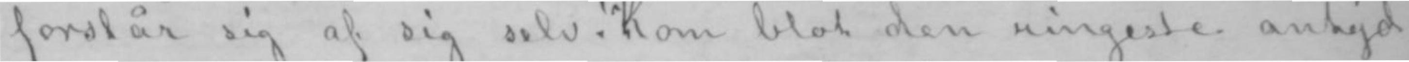forstår sig af sig selv! Kom blot den ringeste antyd-
